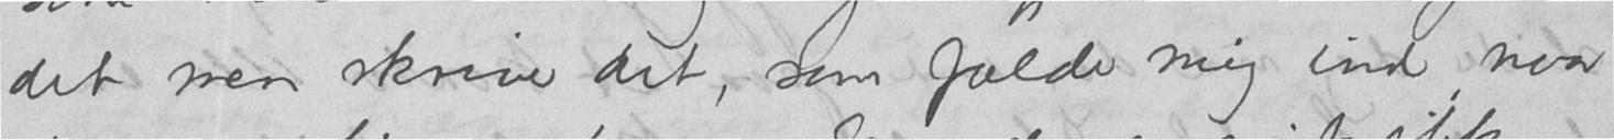det men skriver det, som falder mig ind, naar
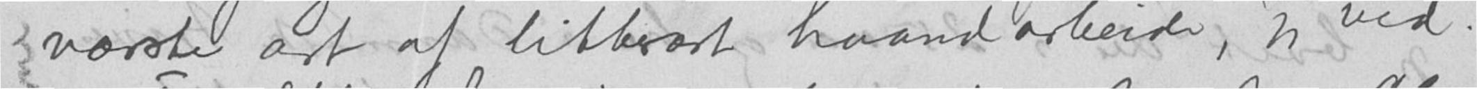værste art af litterært haandarbeide, jeg ved.
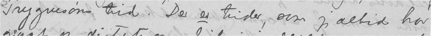Trygvesøn tid. Der er tider, som jeg altid har
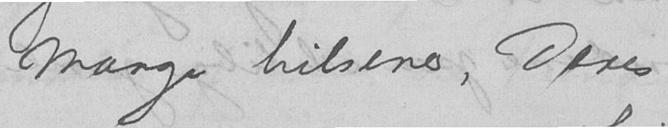Mange hilsener, Deres
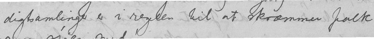digtsamlinger er i regelen til at skræmme folk
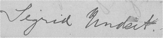Sigrid Undset.
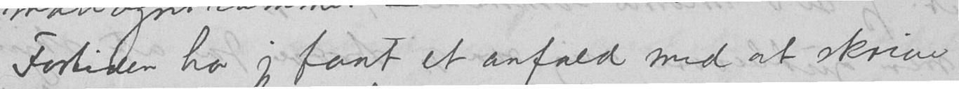Fortiden har jeg faat et anfald med at skrive
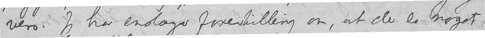vers. Jeg har enslags forestilling om, at der er noget
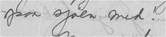paa sjøen med!
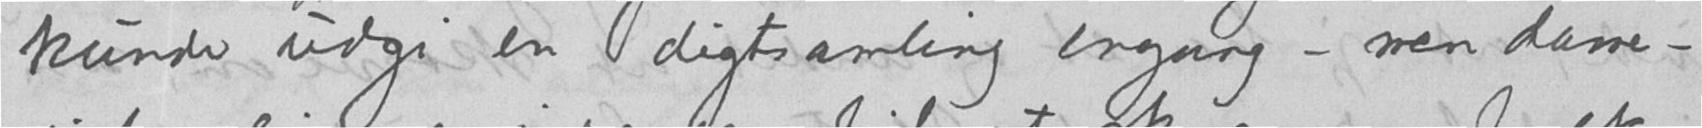kunde udgi en digtsamling engang – men dame -
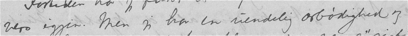vers igjen. Men jeg har en uendelig ærbødighed og
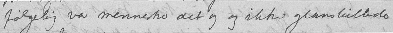følgelig var mennesker det og og ikke glansbilleder
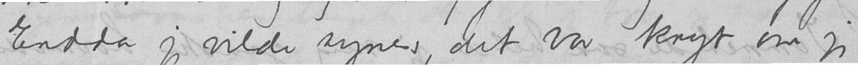Endda jeg vilde synes, det var kryt om jeg
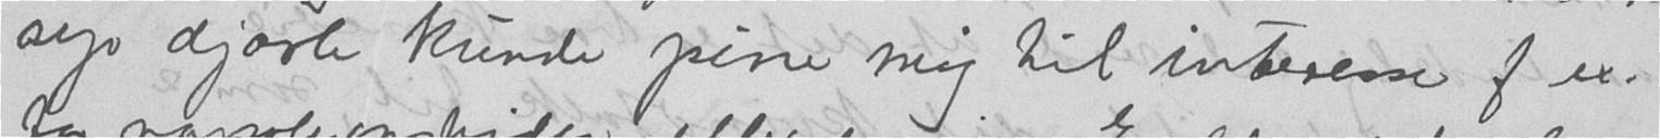syv djævle kunde pine mig til interesse f ex.
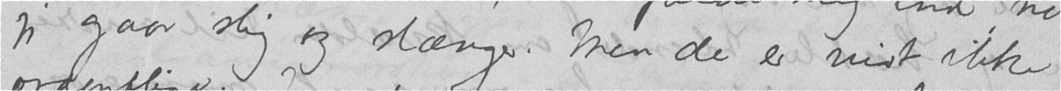jeg gaar slig og slænger. Men de er vist ikke
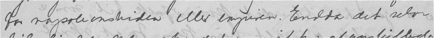for napoleonstiden eller empiren. Endda det selv-
